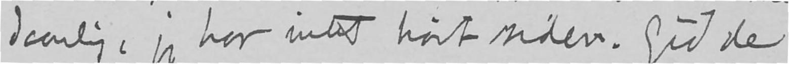daarlig, jeg har intet hørt siden. Gid de
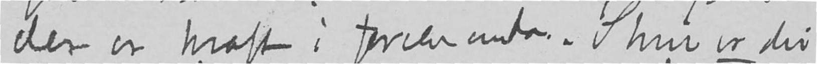der er kraft i farven enda. Skriv er du
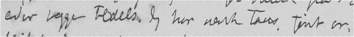eder begge tildels. Jeg har været taus, først ar-
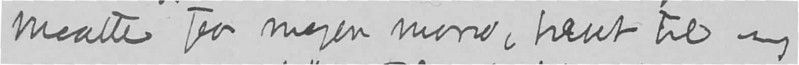maatte faa megen morro, brevet til mig
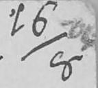16/8
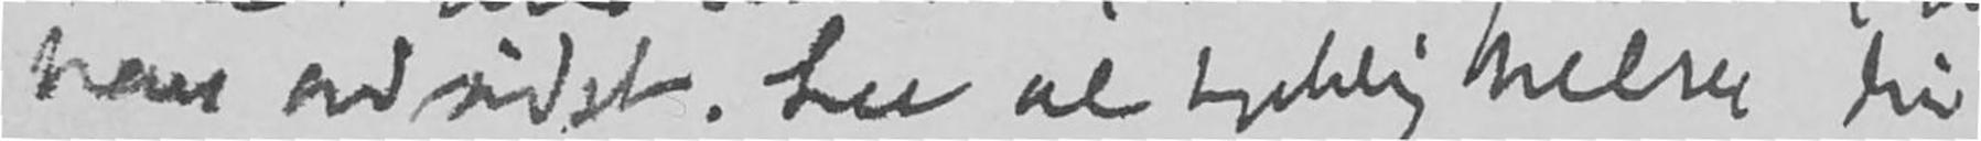hans ord sidst. Lev vel hjertelig hilsen din
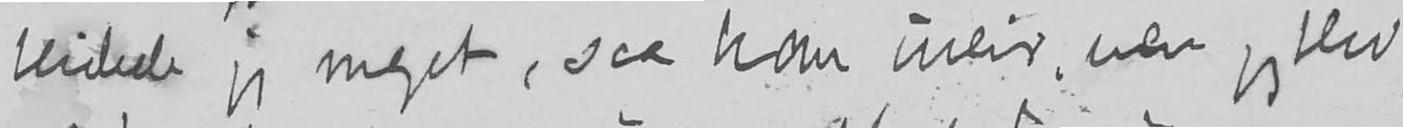beidede jeg meget, saa kom uveir, men jeg blev
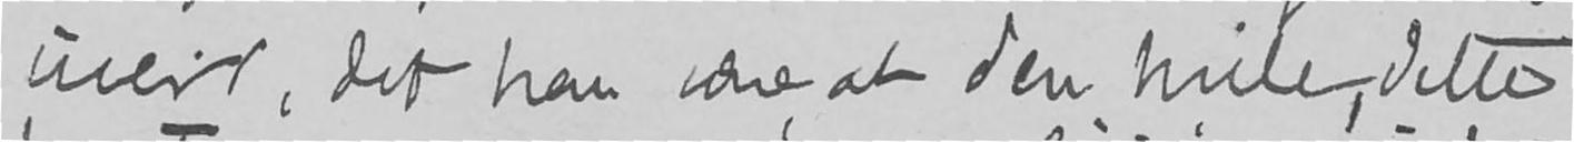uveir, det kan være at den kulde, dette
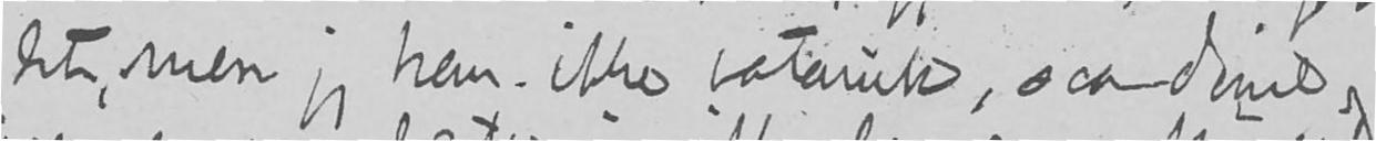det, men jeg kan ikke botanik, saa dine
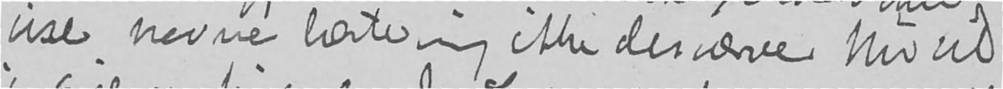vise navne lærte mig ikke desværre. Nu vil
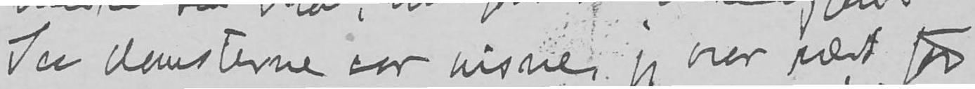Ja blomsterne var visne, jeg var ræd for
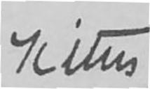Kitty
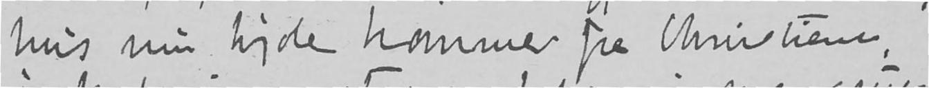hvis nu kjole kommer fra Christiania,
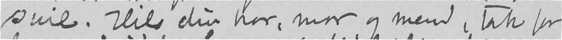snil. Hils din bror, mor og mand, tak for
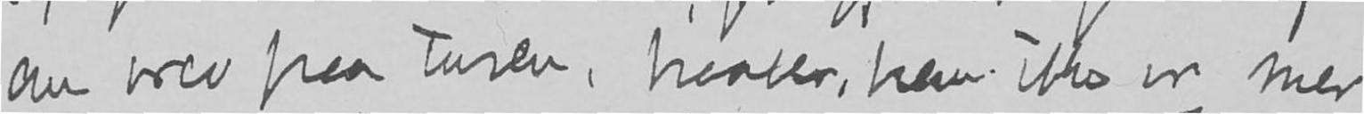om brev paa turen, haaber, han ikke er mer
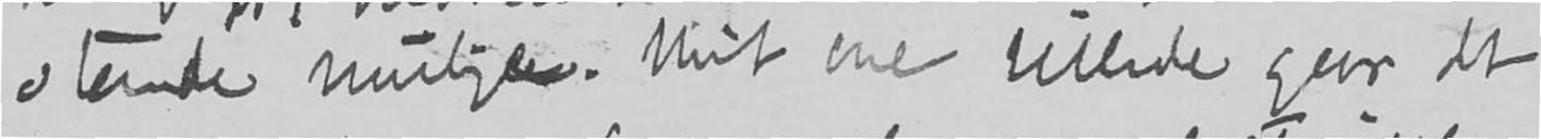stande mulige. Mit ene billede gaar det
